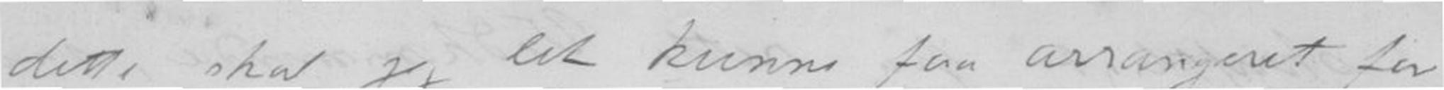dette skal jeg let kunne arrangeret for
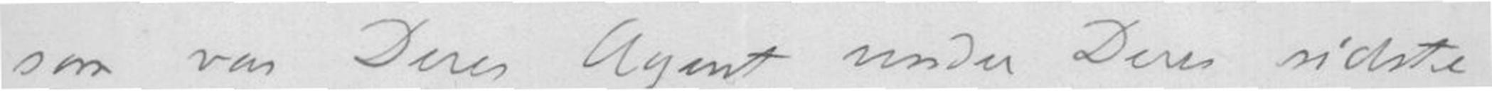som var Deres Agent under Deres sidste
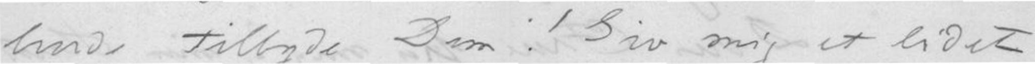burde tilbyde Dem! Giv mig et lidet
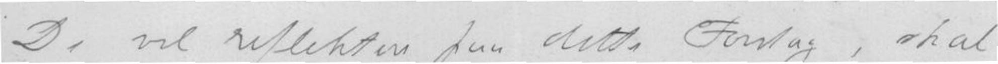De vil reflektere paa dette Forslag, skal
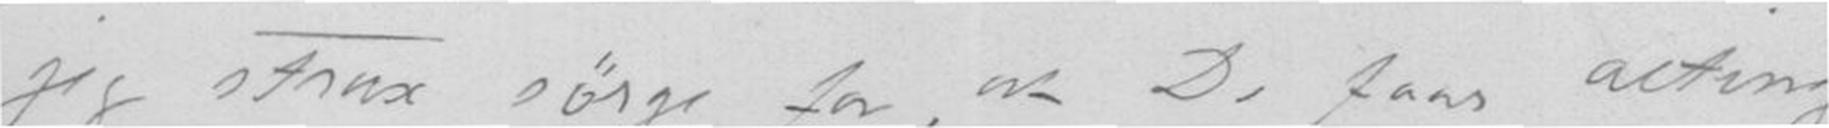jeg strax søge for, at De faar alting
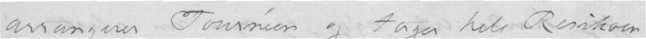arrangerer Tournéen og tager hele Risikoen,
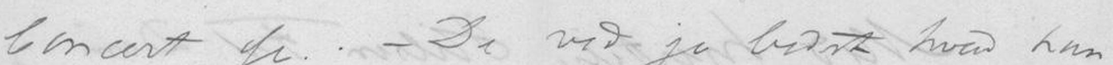Corncert osv. - De ved jo bedst, hvad han
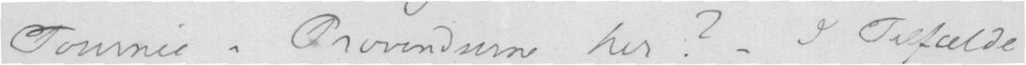Tournée i Provindserne her? - I Tilfælde
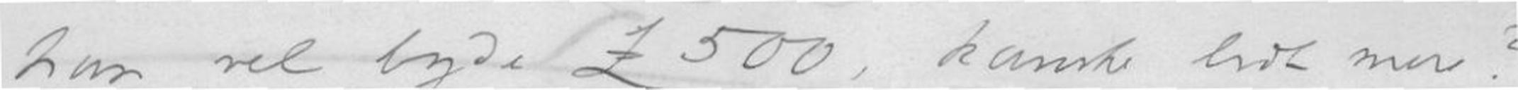han vel byde £500, kanske lidt mer?
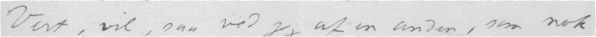Vert, vil, saa ved jeg af en anden, som nok
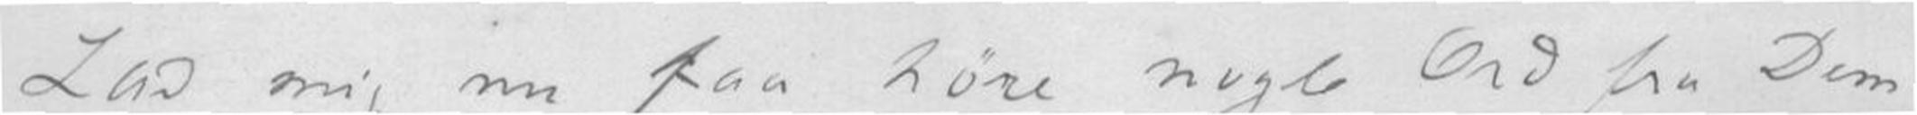Lad mig nu faa høre nogle Ord fra Dem
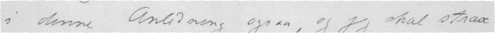i denne Anledning ogsaa, jeg skal strax
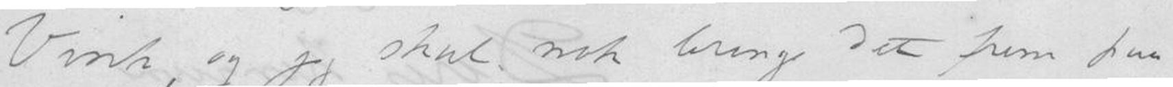Vink, og jeg skal nok bringe det frem paa
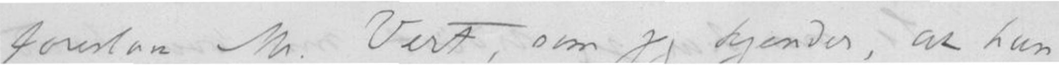forstaa Mr. Vert, som jeg kjender, at han
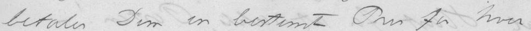betaler Dem en bestemt Pris for hver
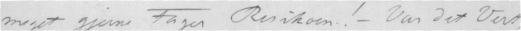meget gjerne tager Risikoen! - Var det Vert
